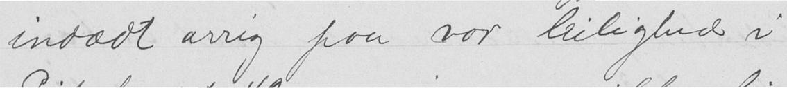indædt arrig paa vor leilighed i
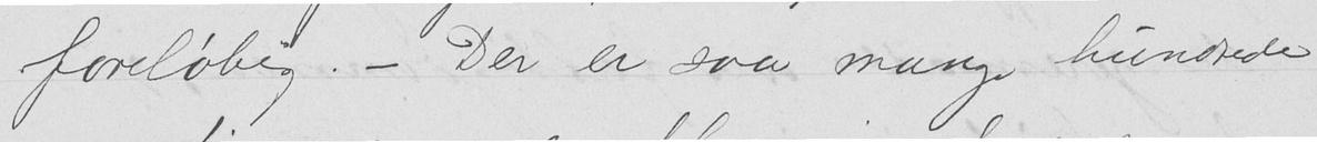foreløbig. - Der er saa mange hundrede
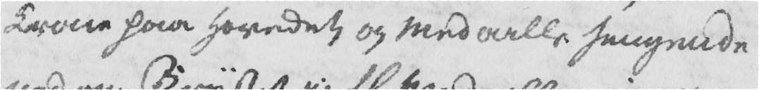Krone paa Hovedet og Medaille hengende
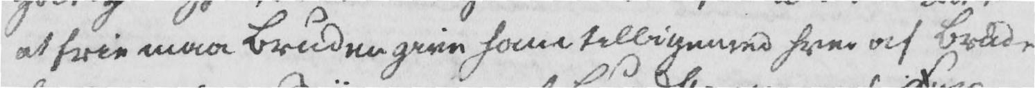at frie maa Bruden give ham tilligemed hver af Brude-
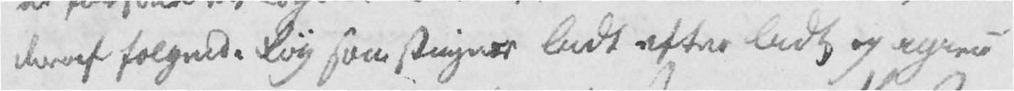deraf følgende Røg som stiger lidt efter lidt og igien-
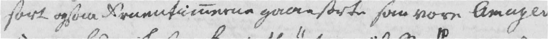sort ogsa Fruentimerne gaae sorte som vore Amager
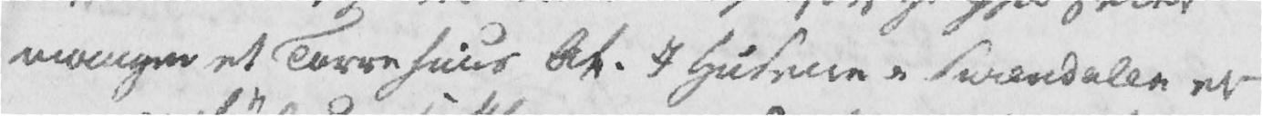mangen et Tørrehuus af. I Husene i Surendalen er
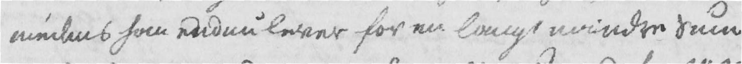medens han endnu lever for en langt mindre Sum
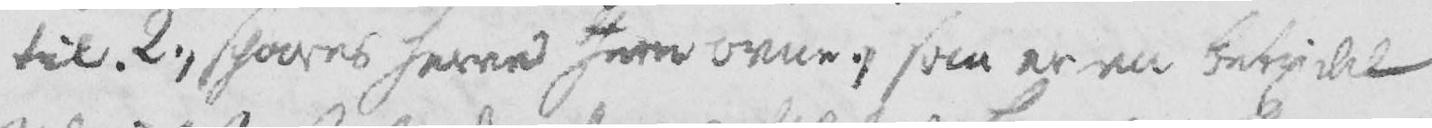til. 2., spares herved Jernovne, som er en betydel
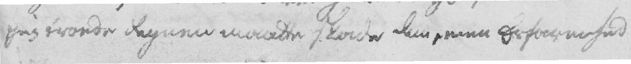Jeg troede Røgen maatte skade dem, men Erfarenhed
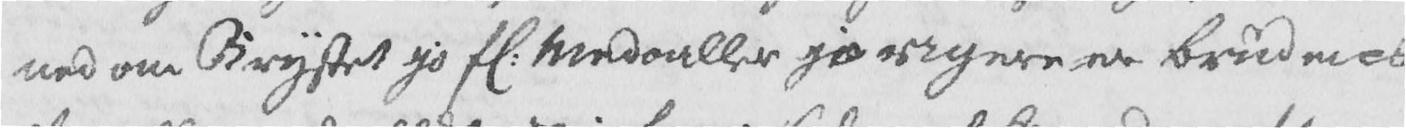ned om Brystet jo fl. Medailler jo rigere er Brudens
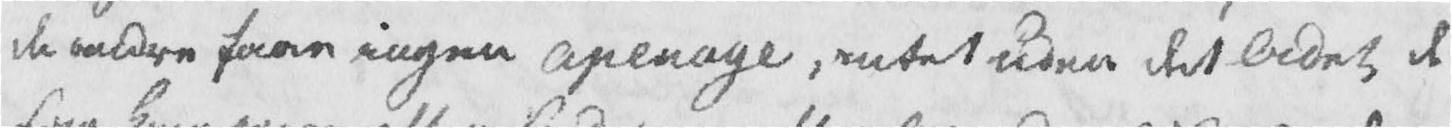de andre faae ingen Apenage, indet uden det lidet de
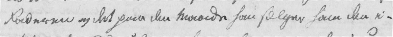Faderen og det paa den Maade han sælger ham den i-
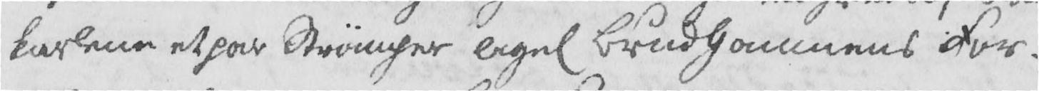karlene et par Strømper ligel Brudgommens For-
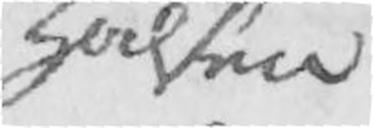Halsen
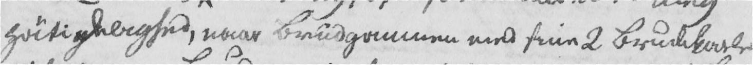Høitigdelighed, naar Brudgommen med sine 2 Brudekarle
